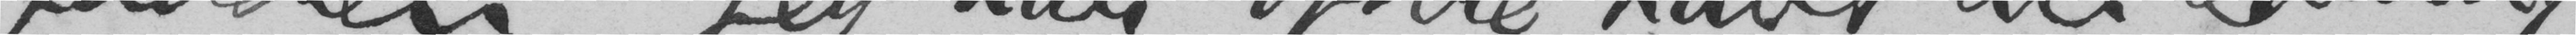faderen. Jeg har oftere havt anledning
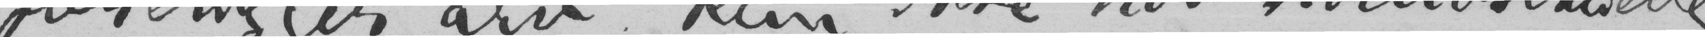foreligger arv, kun ikke hos homosexuelle
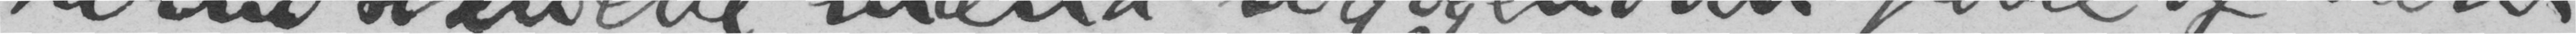homosexuelle mænd sig og endnu færre af dem
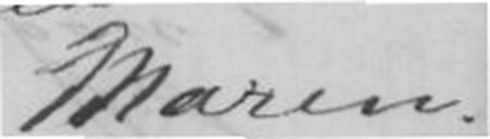Maren.
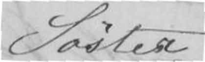Søster
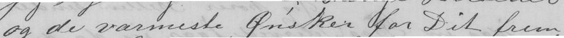og de varmeste Ønsker for Dit frem-
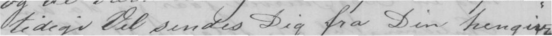tidige Vel sendes Dig fra Din hengivne
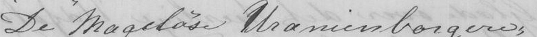De "Mageløse Uranienborgere"
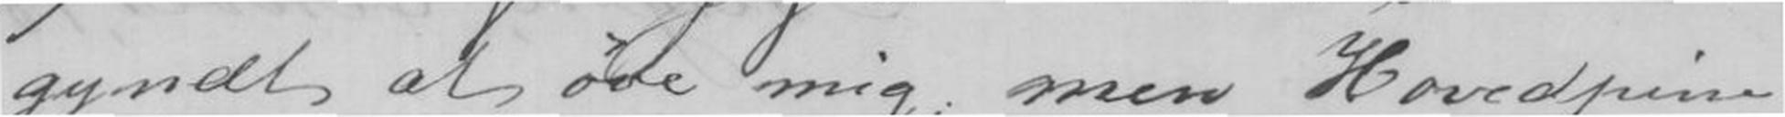gyndt at øve mig, men Hovedpine
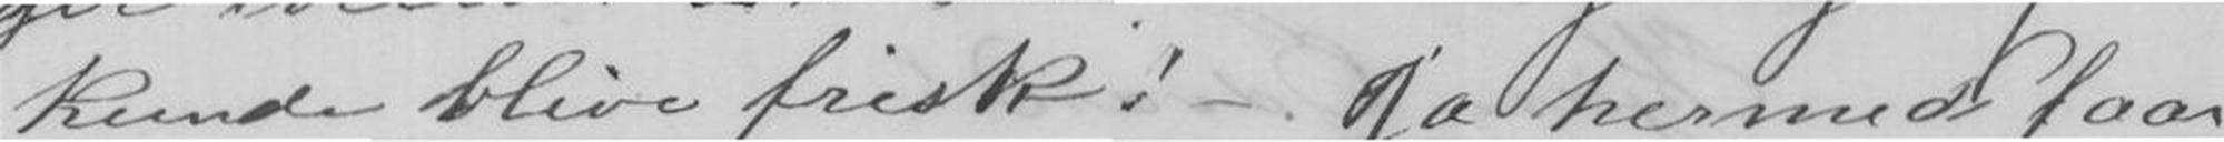kunde blive frisk! - Ja hermed faar
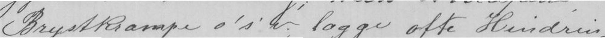Brystkrampe o's'v. lægge ofte Hindring-
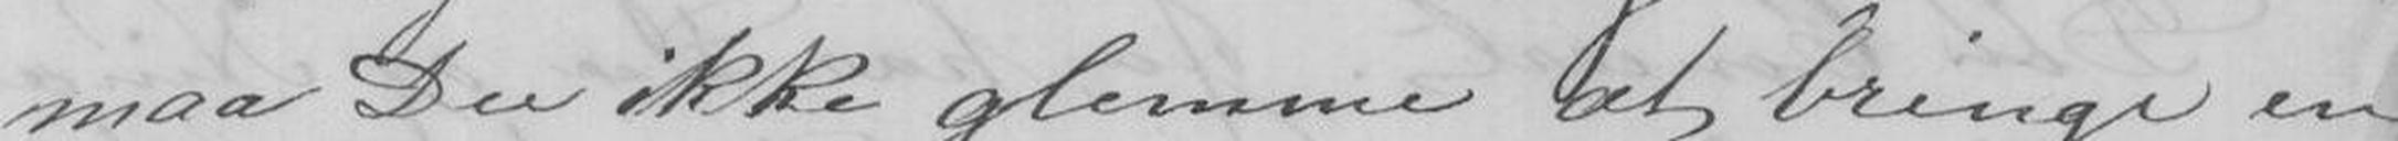maa Du ikke glemme at bringe en
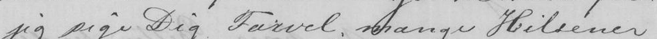jeg sige Dig Farvel; mange Hilsener
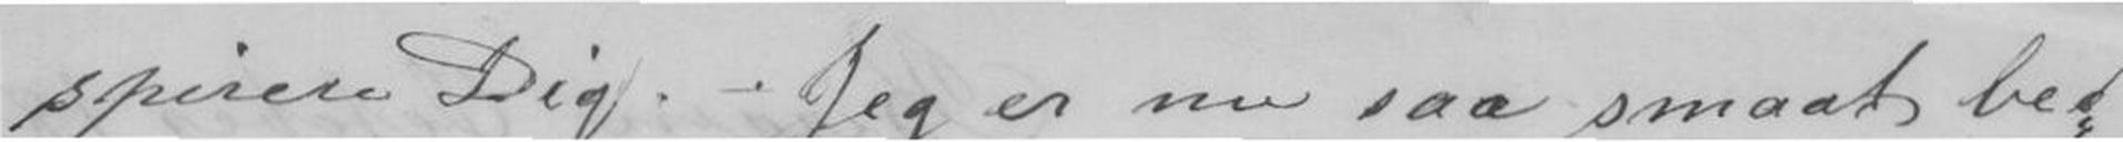spirere Dig. Jeg er nu saa smaat be-
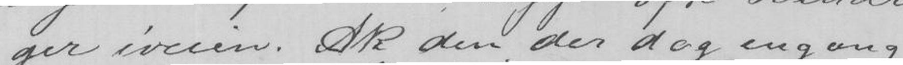ger iveien. Ak den der dog engang
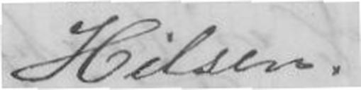Hilsen.
